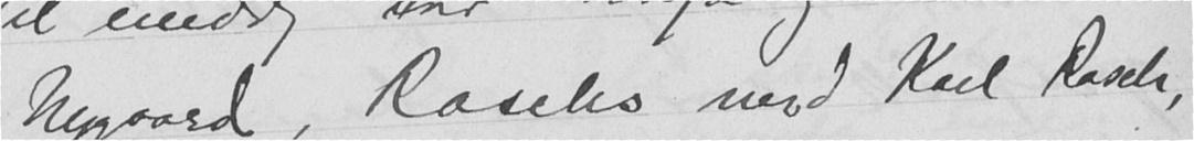Nygaard, Raschs med Karl Rasch,
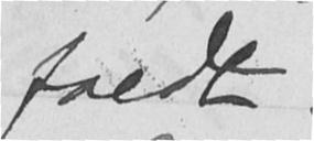faldt.
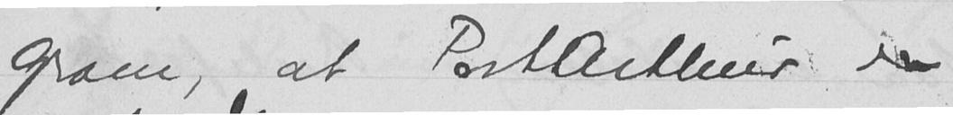gram, at Port Arthur er
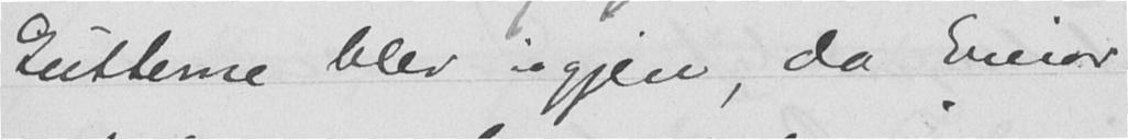Gutterne blev igjen, da Einar
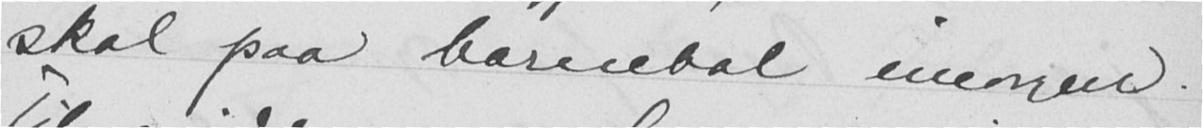skal paa barnebal imorgen.
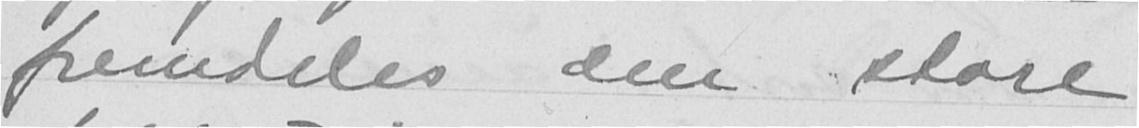fremdeles den store
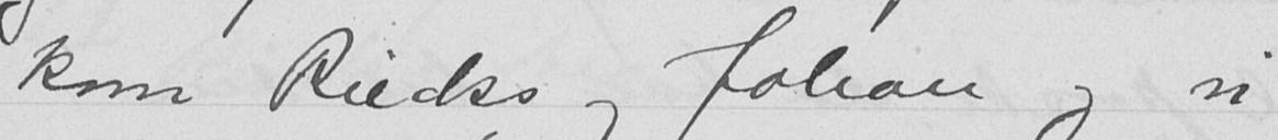kom Riecks og Johan og vi
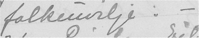folkeuvilje -
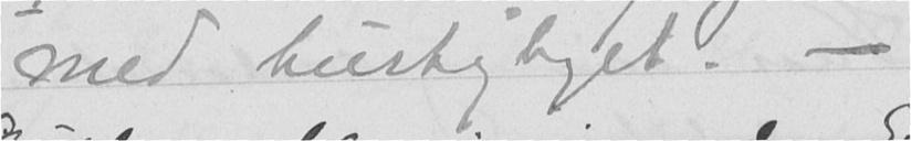med hurtigtoget. -
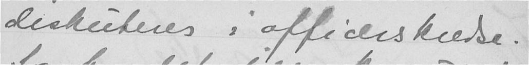diskuteres i officerskredse.
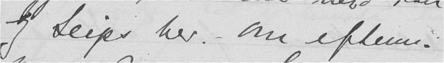og Seips her. Om efterm.
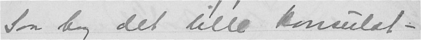Saa bag det lille konsulat-
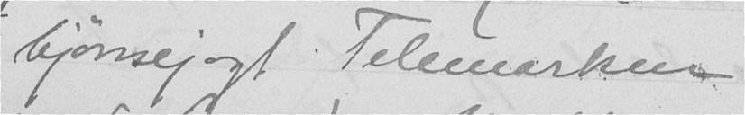bjørnejagt Telemarken
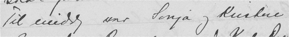Til middag var Sonja og Kristen
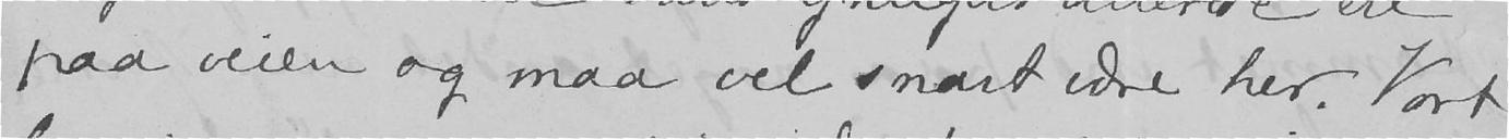paa veien og maa vel snart være her. Vort
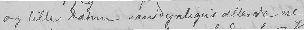og lille Dahm sandsynligvis allerede ere
